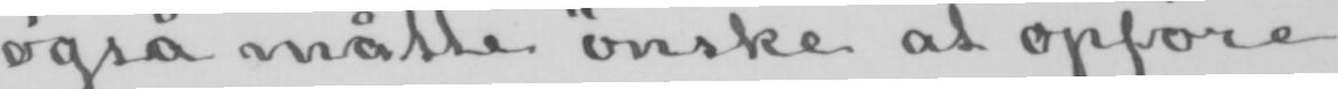også måtte ønske at opføre
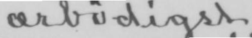ærbødigst
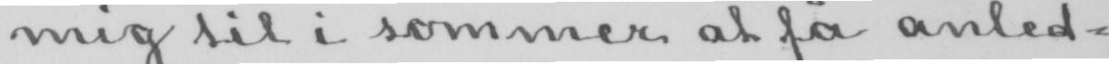mig til i sommer at få anled-
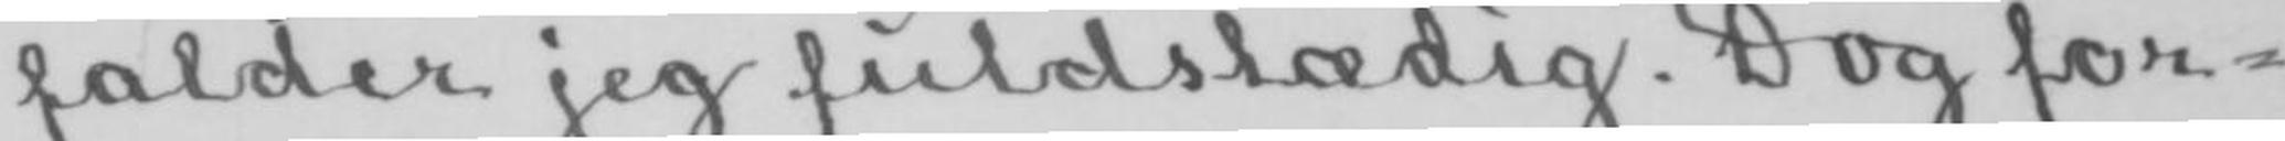falder jeg fuldstædig. Dog for-
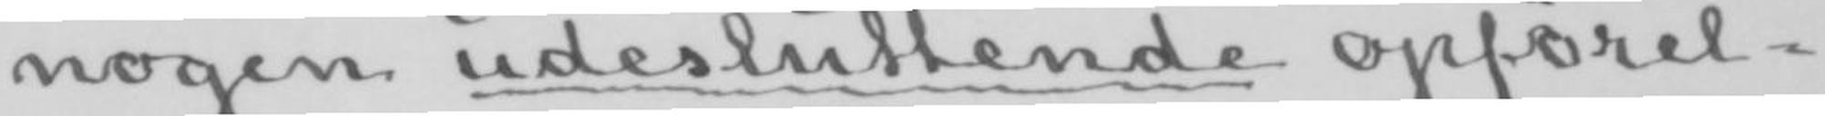nogen udesluttende opførel-
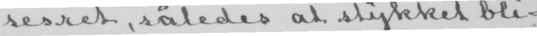sesret, således at stykket bli-
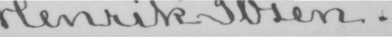Henrik Ibsen.
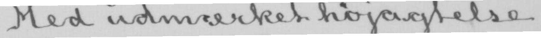Med udmærket højagtelse
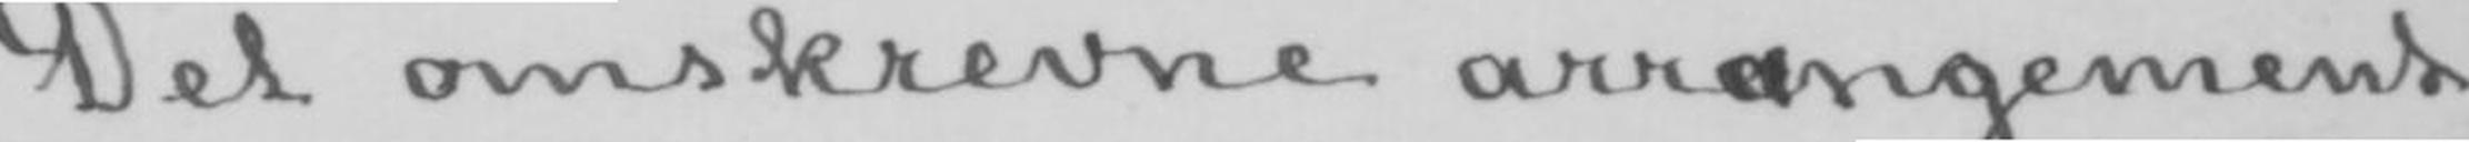Det omskrevne arreangement
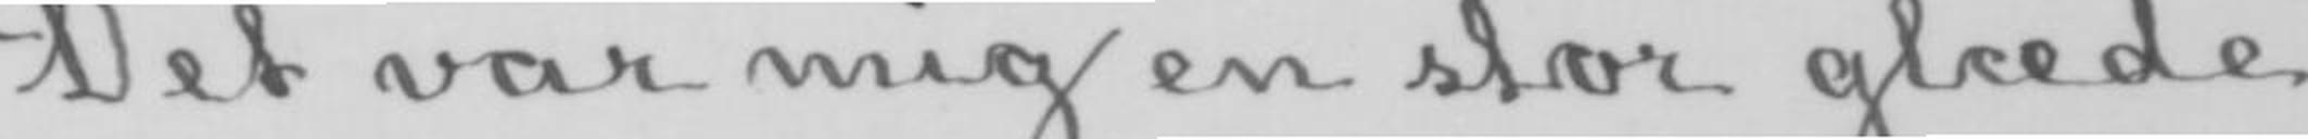Det var mig en stor glæde
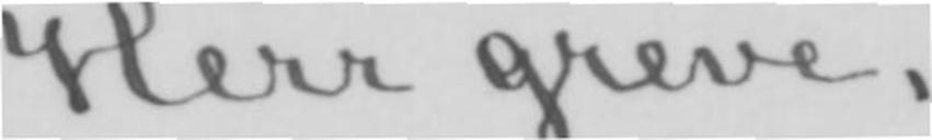Herr greve,
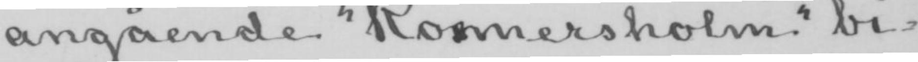angående «Rosmersholm» bi-
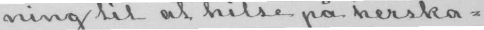ning til at hilse på herska-
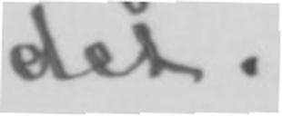det.
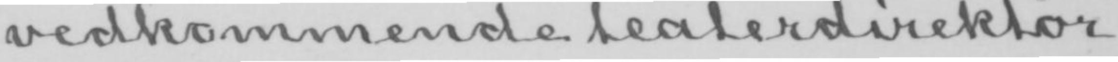vedkommende teaterdirektør
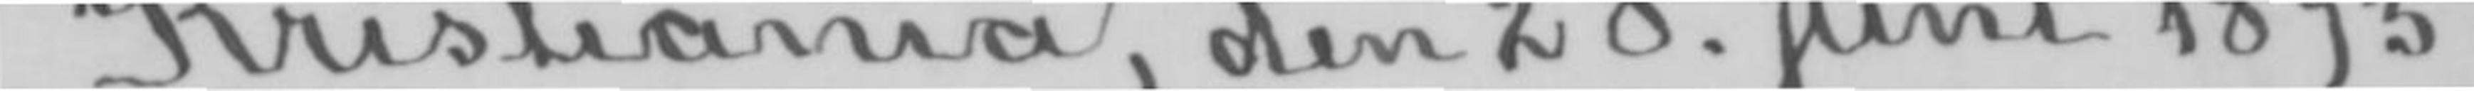Kristiania, den 28. Juni 1893.
